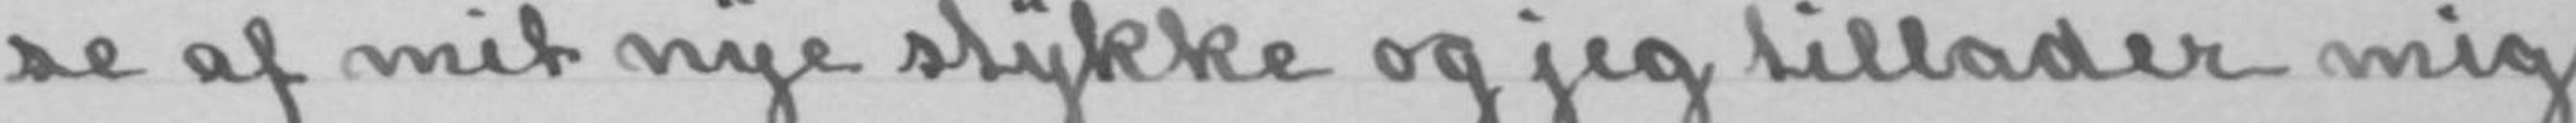se af mit nye stykke og jeg tillader mig
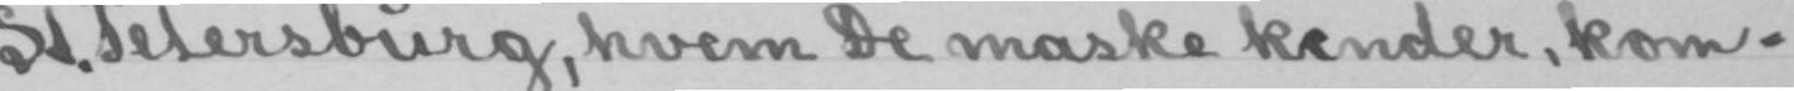St. Petersburg, hvem De måske kender, kom-
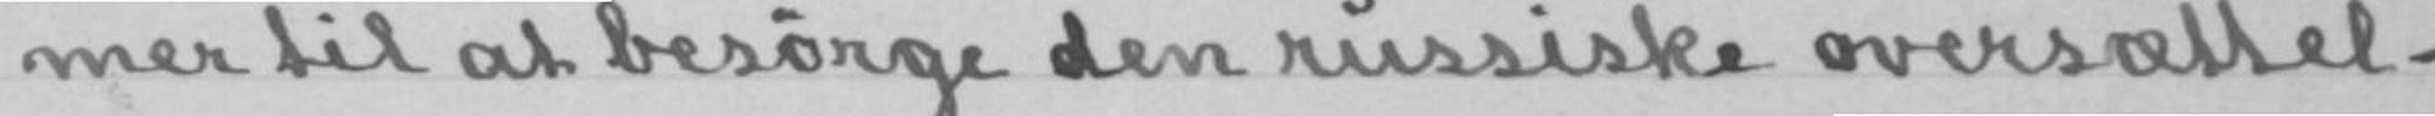mer til at besørge den russiske oversættel-
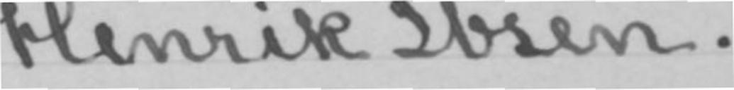Henrik Ibsen.
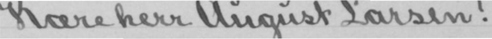Kære herr August Larsen!
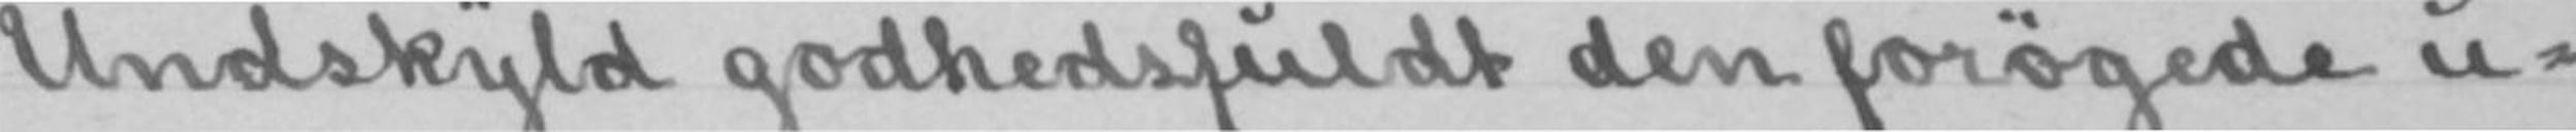Undskyld godhedsfuldt den forøgede u-
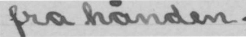fra hånden.
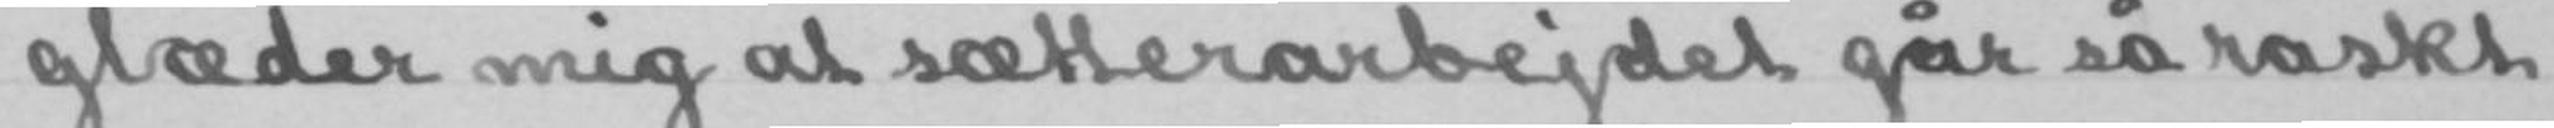glæder mig at sætterarbejdet går så raskt
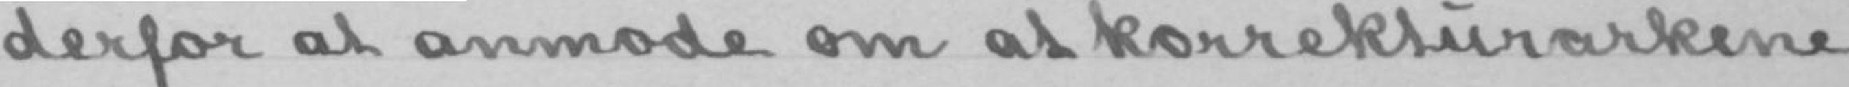derfor at anmode om at korrekturarkene
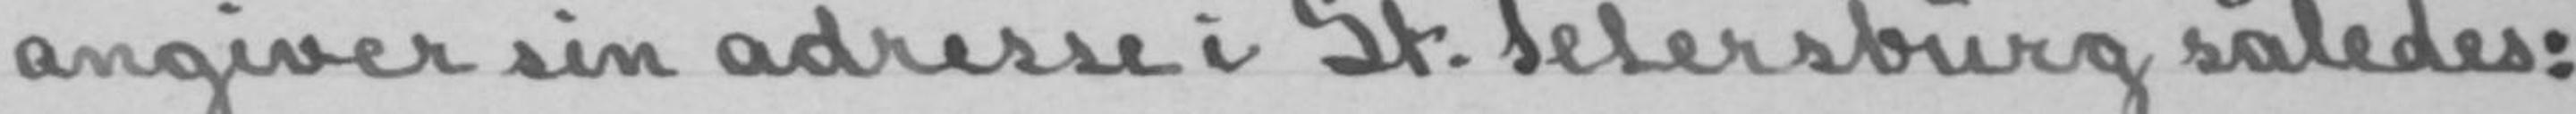angiver sin adresse i St. Petersburg således:
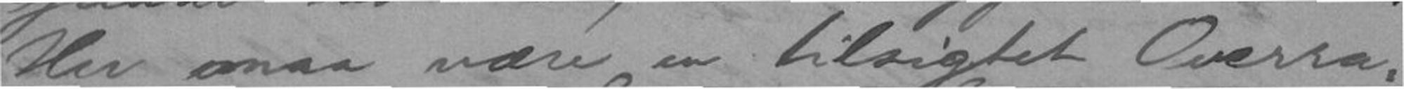Her maa være en tilsigtet Overra-
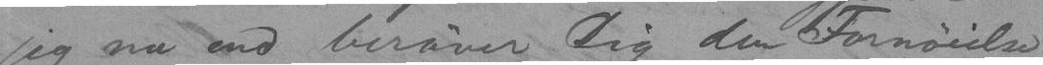jeg nu end berøver Dig den Fornøielse,
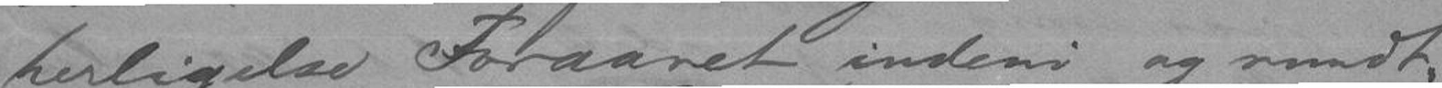herligelse Foraaret indeni og rundt-
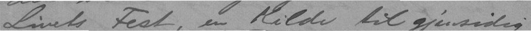Livets Fest, en Kilde til gjensidig
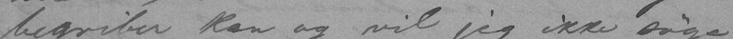begriber kan og vil jeg ikke søge
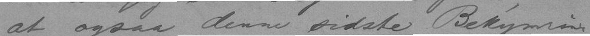at ogsaa denne sidste Bekymrin-
